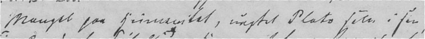Mangel paa Humanitet, uagtet Plato selv i sin
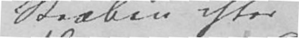Stræben efter
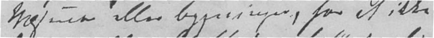Væsener eller Bygninger, for at ikke
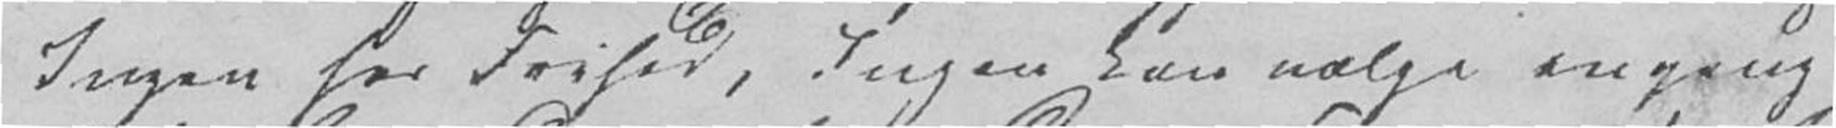Ingen har Frihed, Ingen kan vælge engang
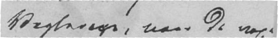Vogterne, naar de voxe-
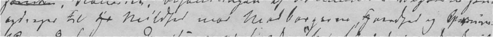opdrages til Mildhed mod Medborgerne, Haardhed og XXX-
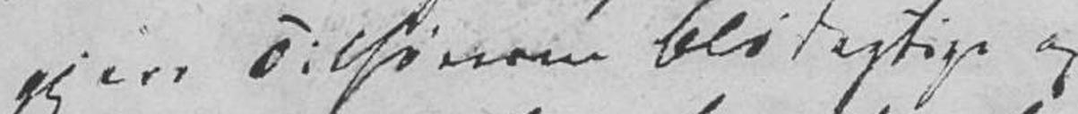gjøre Tilhørerne blødagtige og
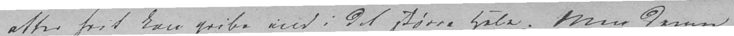atter frit kan gribe ind i det større Hele. Men denne
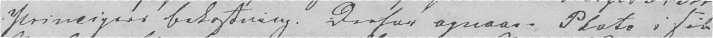Principers Bekostning. Derfor opnaar Plato i sin
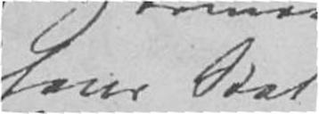hans Stat
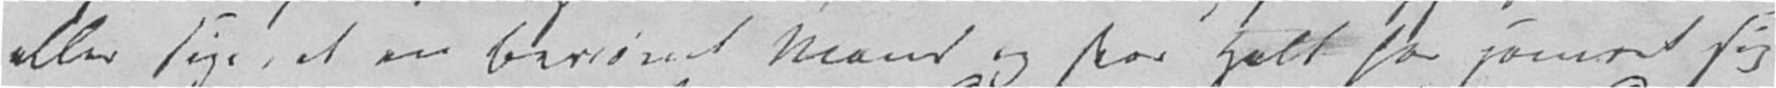eller sige, at en berømt Mand og stor Helt har jamret sig
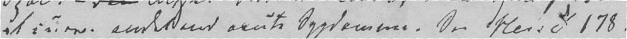at curere andet end acute Sygdomme. See Slar 178.
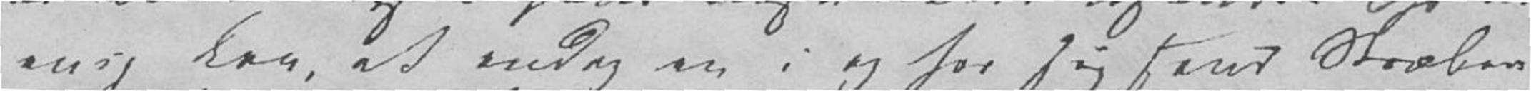evig Lov, at endog en i og for sig sand Stræben,
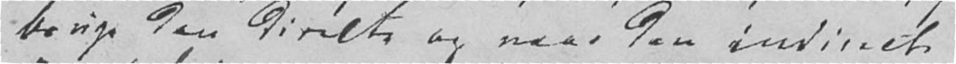bruge den directe og naar den indirecte
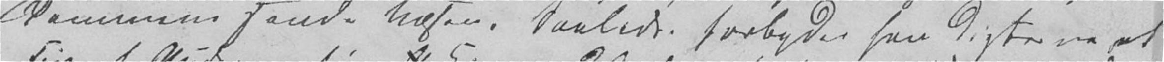dommens sande Væsen. Saaledes forbyder han Digterne at
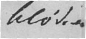bløde
